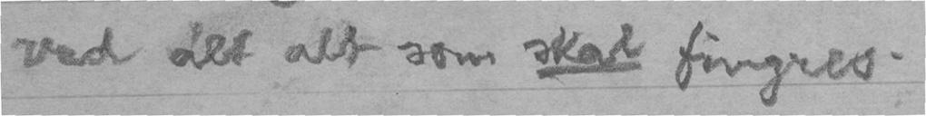ved alt alt som skal fingres.
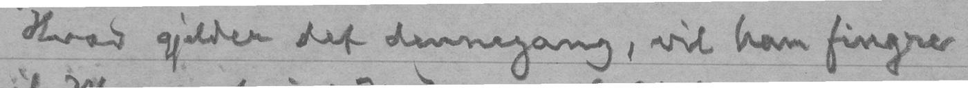Hvad gjelder det dennegang, vil han fingre
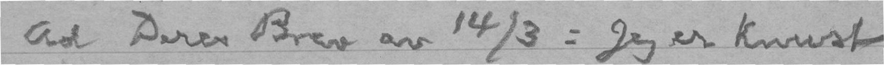Ad Deres Brev av 14/3: Jeg er knust
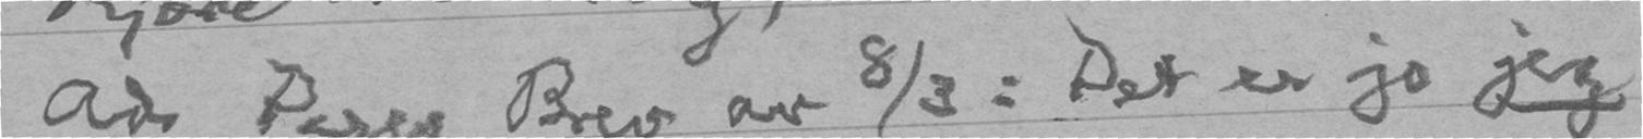Ad Deres Brev av 8/3: Det er jo jeg
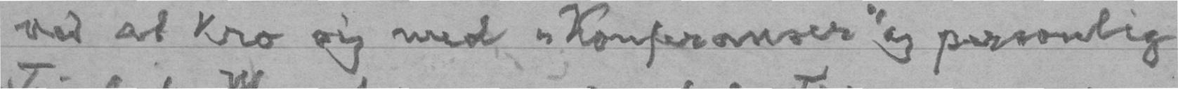ved at kro sig med "Konferanser" og personlig
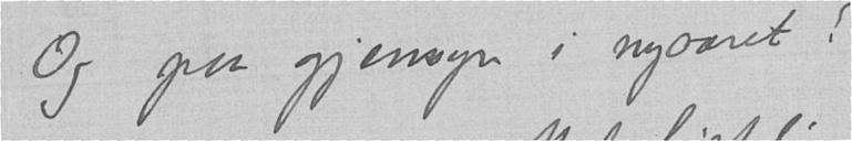Og paa gjensyn i nyaaret!
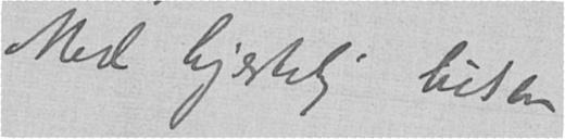Med hjertelig hilsen
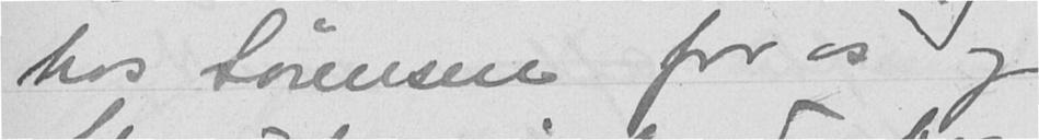hos Sørensen for os og
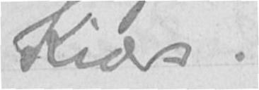Kiærs.
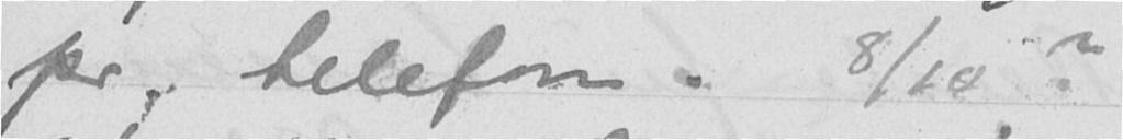pr. telefon. 8/10 ?
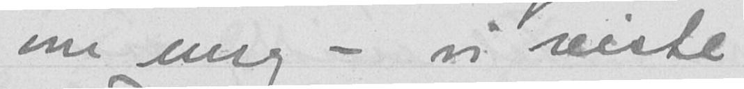om mrg - vi reiste
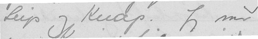Seip og Knap. Jeg var
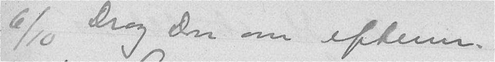6/10 Drog Don om efterm.
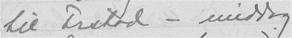til Frstad - middag
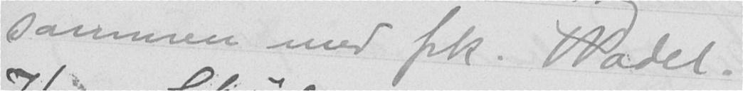sammen med frk. Wadel.
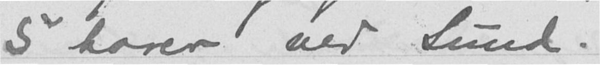5 harer ved Sund.
